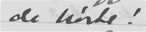de hørte!
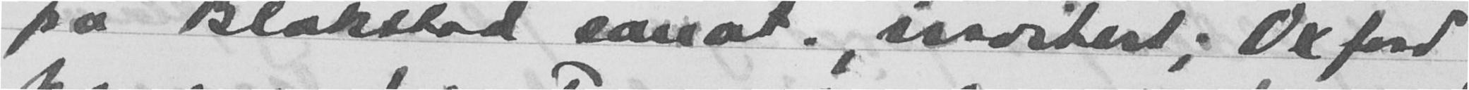på Blakstad sanat. invitert; Oxford
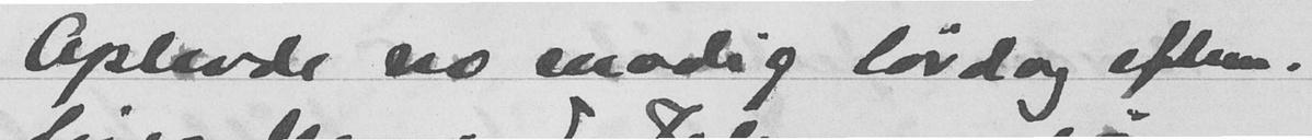Oplevde no modig lørdag efterm.
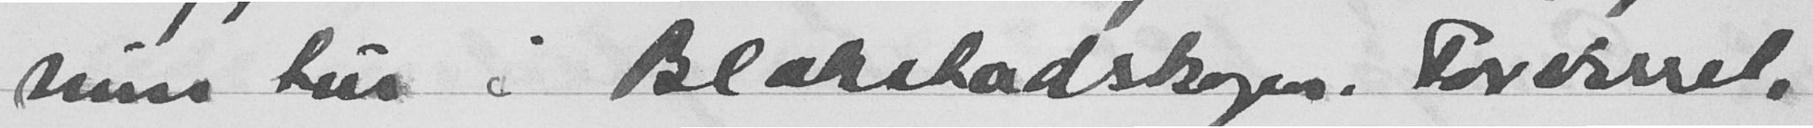min tur i Blakstadskogen. Forvirret,
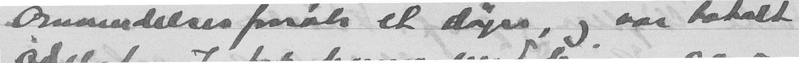omvendelsesforøk et døgn, og var totalt
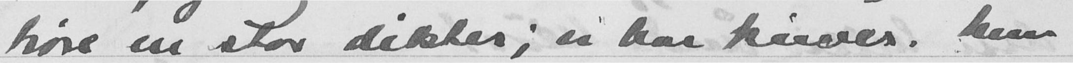høre en stor dikter; vi har trinser. Kun
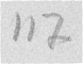117
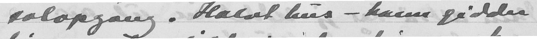solopgang. Halvt hus - hvem gidder
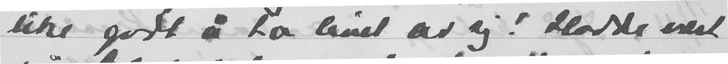like godt å ta livet av sig! Hadde vært
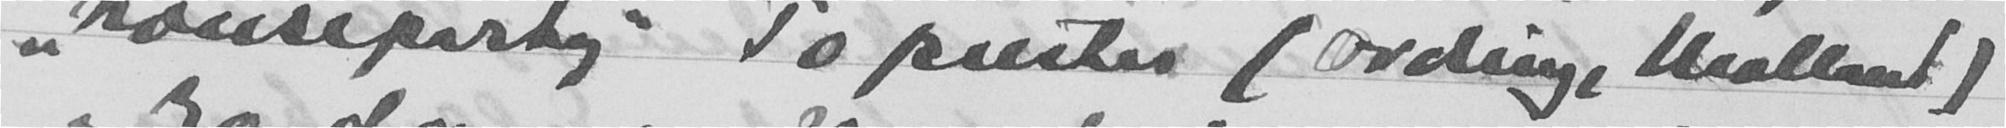"houseparty". To prester (Ording, Mallant)
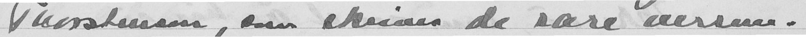Thorstensen, som skriver de sære versene.
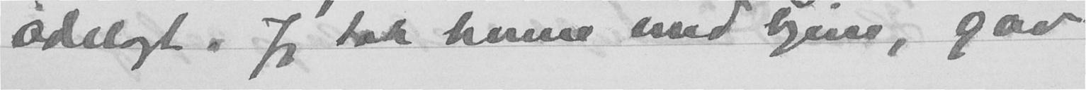ødelagt. Jeg tok henne med hjem, gav
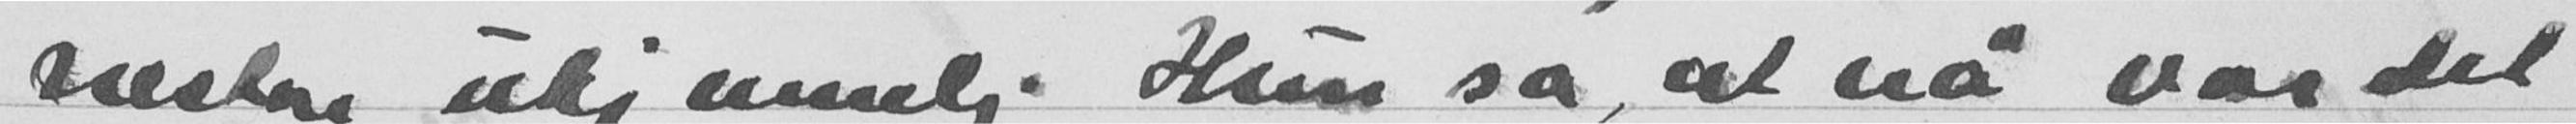nesten ukjennelig. Hun sa, at nå var det
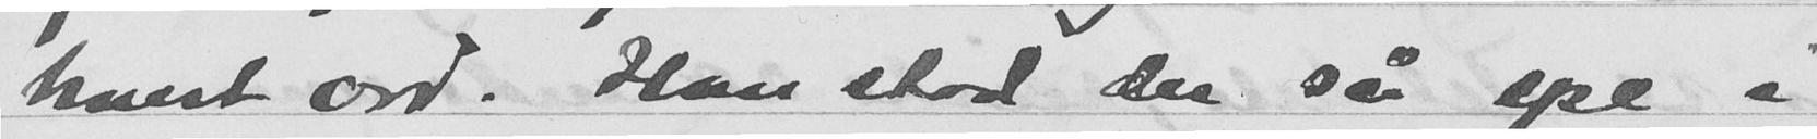hvert ord. Han stod der så spe i
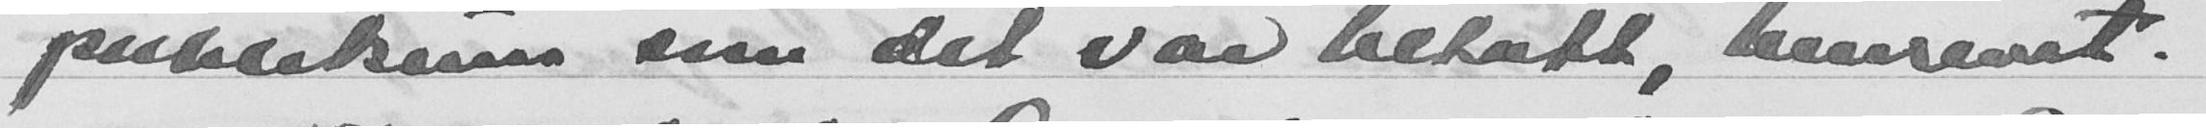publikum som det var betatt, henrevet.
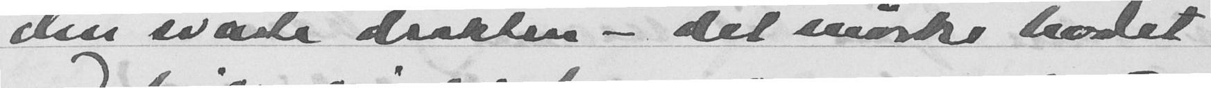den svarte drakten - det mørke hodet
